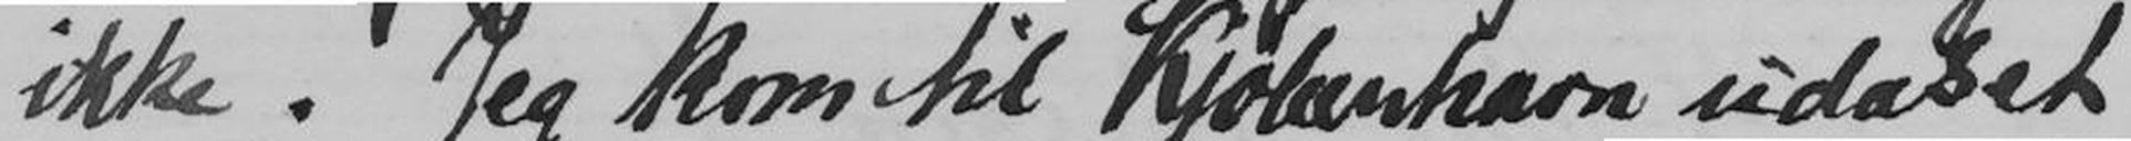ikke. Jeg kom til Kjøbenhavn udaset
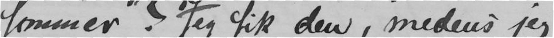sommer? Jeg fik den, medens jeg
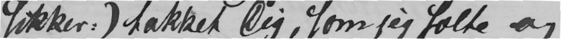sikker:) takket Dig, Som jeg følte og
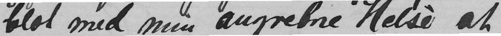blot med min angrebne Helse at
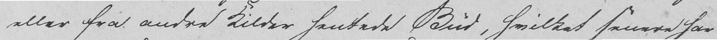eller fra andre Kilder hentede Bud, hvilket senere har
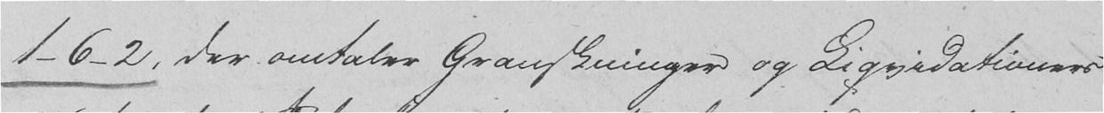1-6-2, der omtaler Granskninger og Liqvidationers
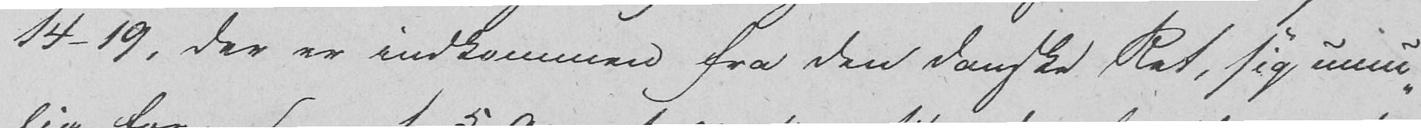14-19, der er indkommen fra den danske Ret, sig umu-
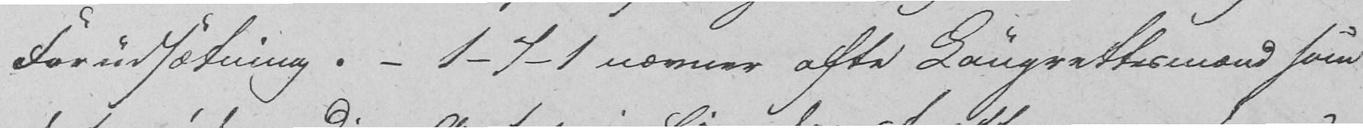Forudsætning. - 1-7-1 nævner ofte Laugrettesmænd som
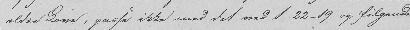ældre Love, passe ikke med det ved 1-22-19 og følgende
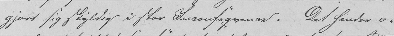gjort sig skyldig i stor Inconseqvence. Det hænder o-
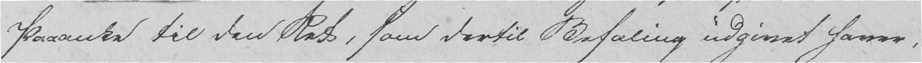Paaanke til den Ret, som dertil Befaling udgivet haver,
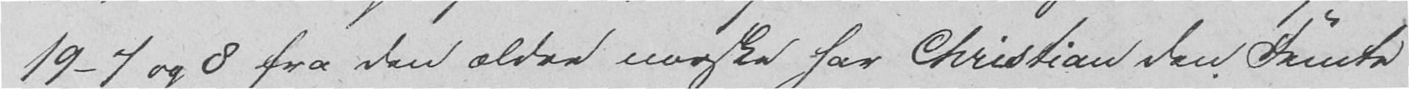19-7 og 8 fra den ældre norske har Christian den Femte
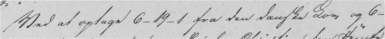Ved at optage 6-19-1 fra den danske Lov og 6-
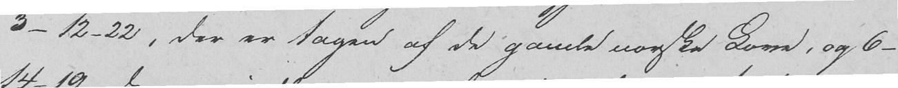3-12-22, der er tagen af de gamle norske Love, og 6-
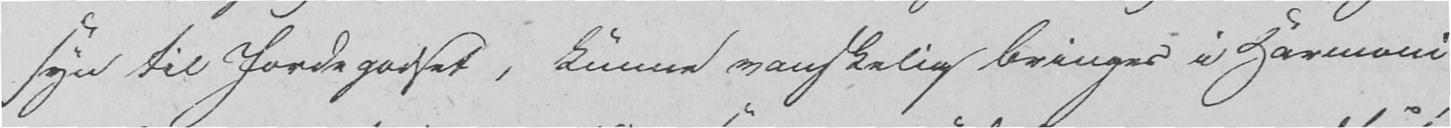syn til Jordegodset, kunne vanskelig bringes i Harmoni
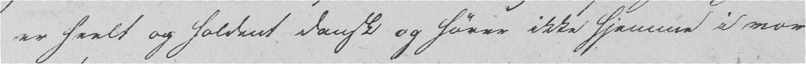er heelt og holdent dansk og hører ikke hjemme i vor
